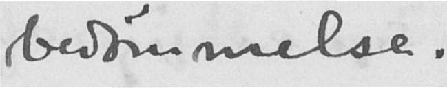bedømmelse.
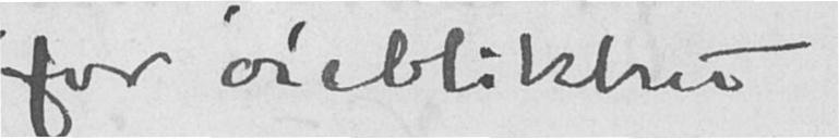for øieblikket
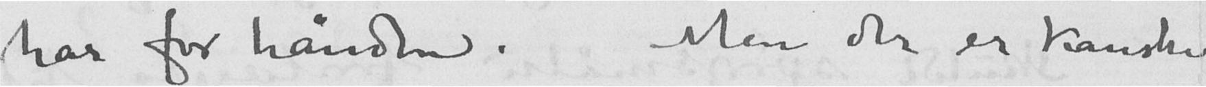har for hånden. Men der er kanske
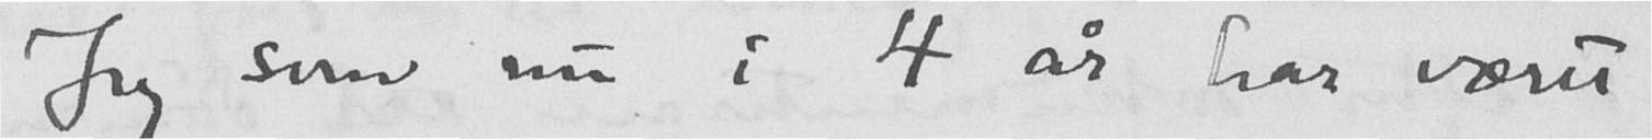Jeg som nu i 4 år har været
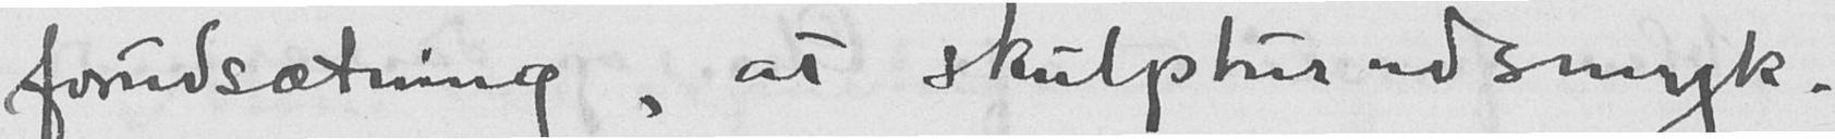forudsætning, at skulpturudsmyk-
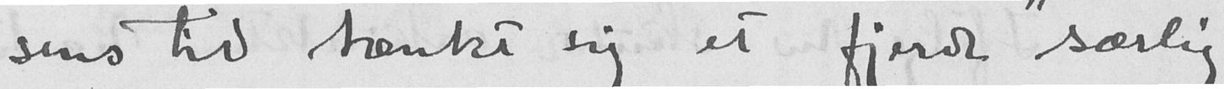sens tid tænkt sig et fjerde "særlig
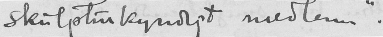skulpturkyndigt medlem".
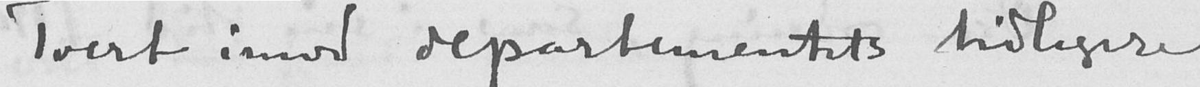Tvert imod departementets tidligere
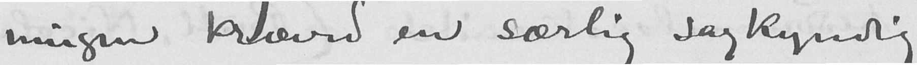ningen kræved en særlig sagkyndig
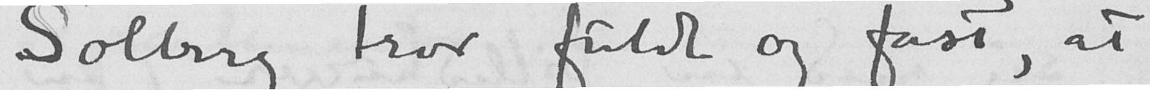Solberg tror fuldt og fast, at
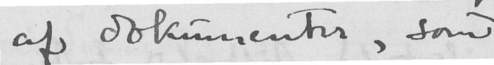af dokumenter, som
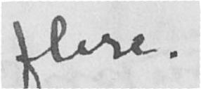flere
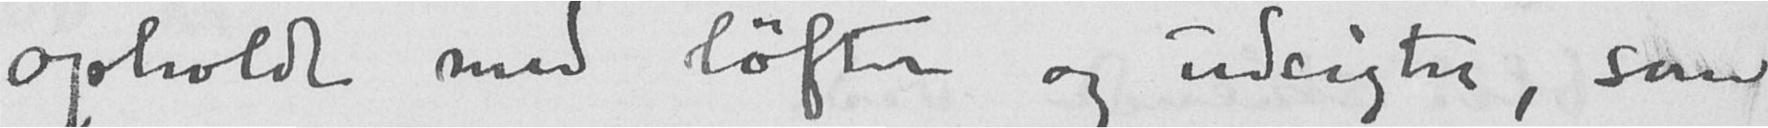opholdt med løfter og udsigter, som
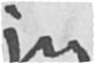jeg
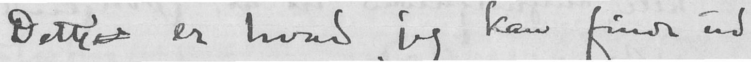Dette er hvad jeg kan finde ud
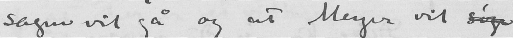sagen vil gå og at Meyer vil sige
